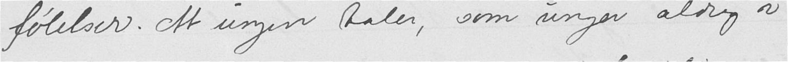følelser. At ungen taler, som unger aldrig i
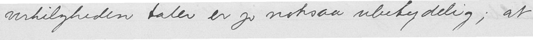virkeligheden taler er jo noksaa ubetydelig; at
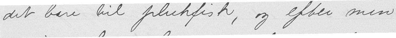det bare til plukfisk, og efter min
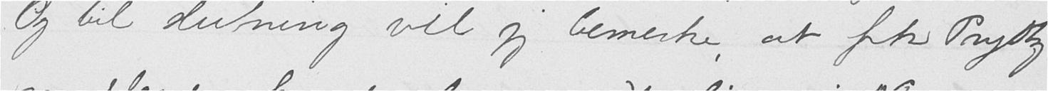Og til slutning vil jeg bemerke, at frk Prydz
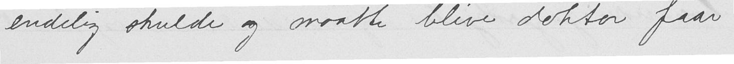endelig skulde og maatte blive doktor faar
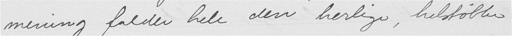mening falder hele den herlige, helstøbte
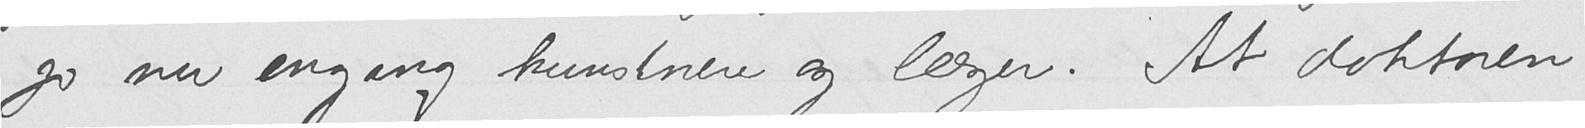jo nu engang kunstnere og læger. At doktoren
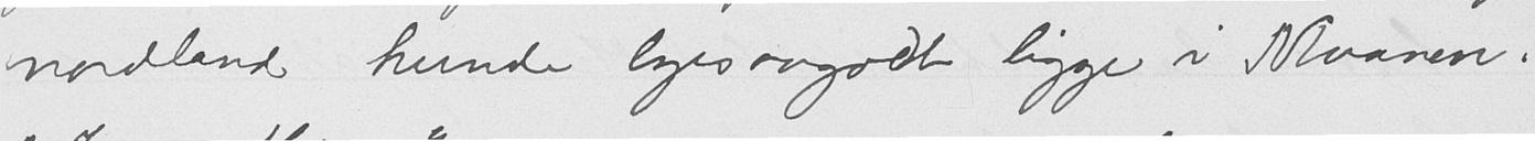Nordland kunde ligesaagodt ligge i Maanen.
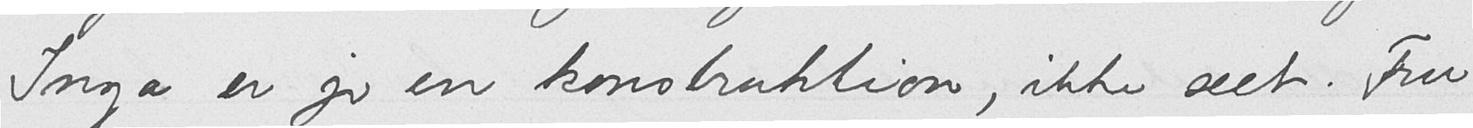Inga er jo en konstruktion, ikke seet. Fru
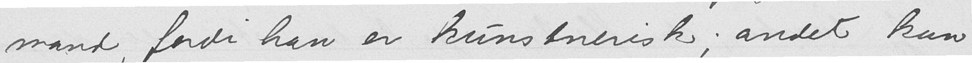mand, fordi han er kunstnerisk; andet kan
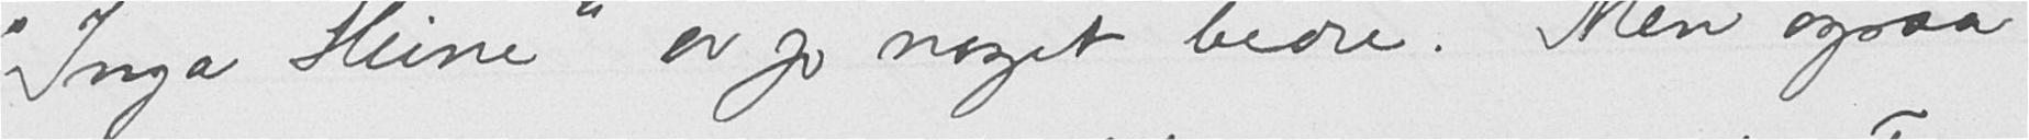"Inga Heine" er jo noget bedre. Men ogsaa
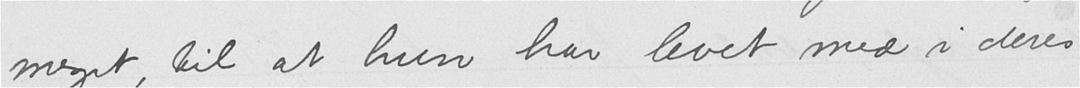meget, til at hun har levet med i deres
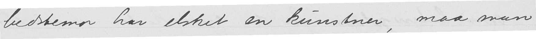bedstemor har elsket en kunstner, maa man
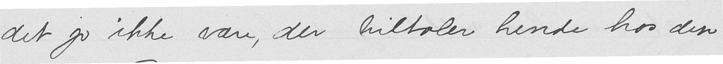det jo ikke være, der tiltaler hende hos den
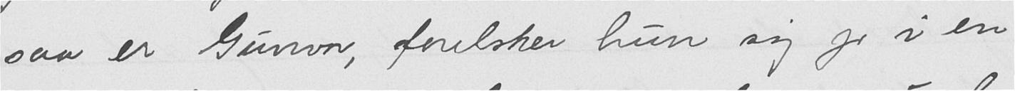saa er Gunvor, forelsker hun sig jo i en
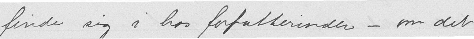finde sig i hos forfatterinder - om det
